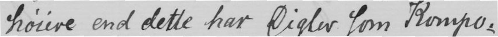høiere end dette har Digter som Kompo-
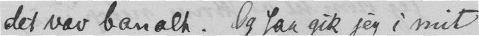det var banalt. Og saa gik jeg i mit
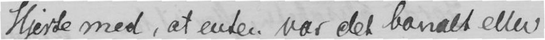Hjerte med, at enten var det banalt eller
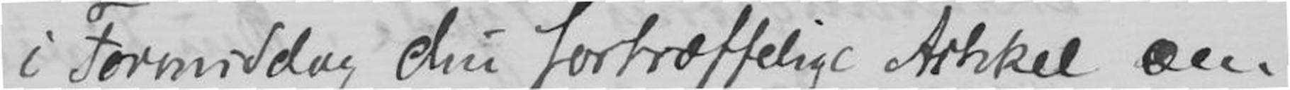i Formiddag din fortræffelige Artikel om
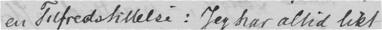en Tilfredstillelse: Jeg har altid likt
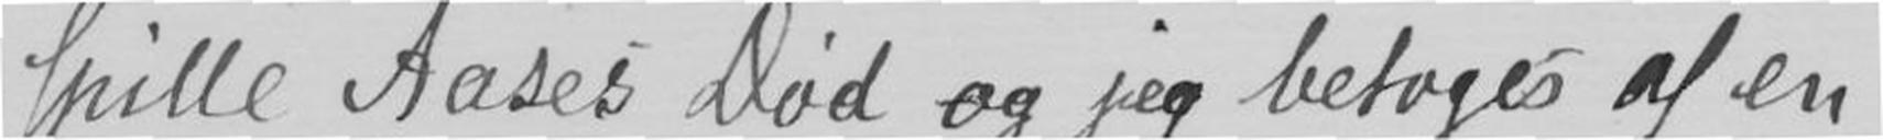spille Aases Død og jeg betages af en
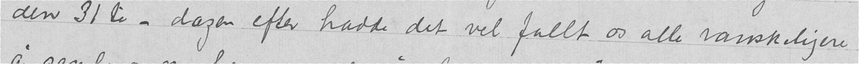den 31te – dagen efter hadde det vel fallt os alle vanskeligere
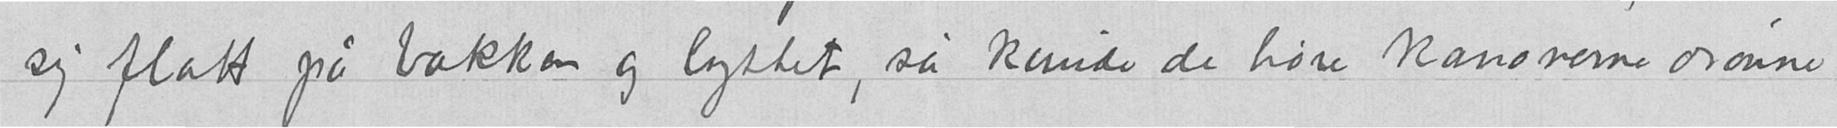sig flatt på bakken og lyttet, så kunde de høre kanonerne drønne
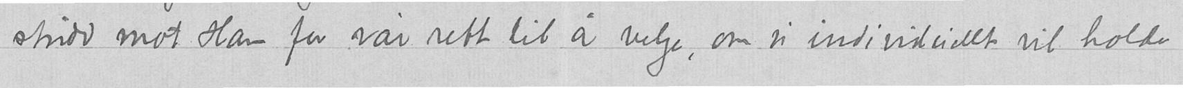stridd mot Ham for vår rett til å velge, om vi individuellt vil holde
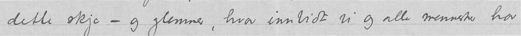dette skje – og glemmer, hvor innbidt vi og alle mennesker har
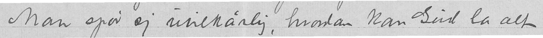Man spør sig uvilkårlig, hvordan kan Gud la alt
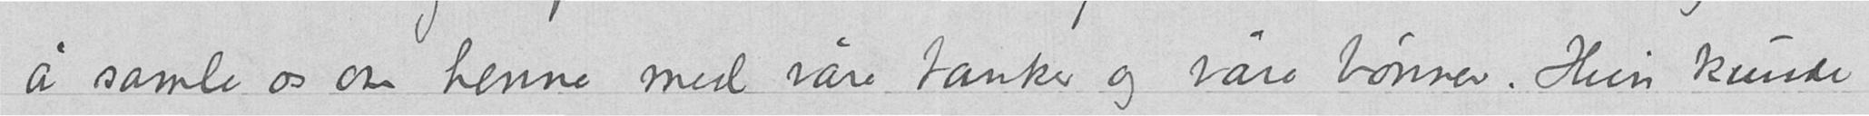å samle os om henne med våre tanker og våre bønner. Hun kunde
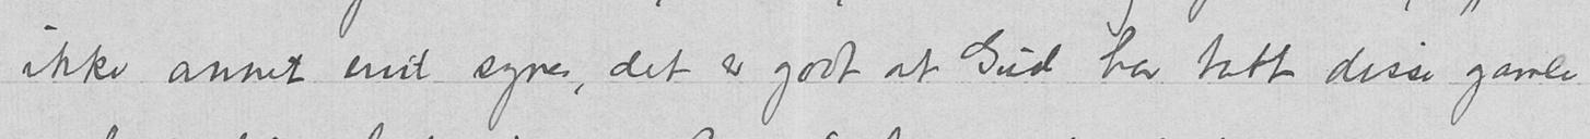ikke annet enn synes, det er godt at Gud har tatt disse gamle
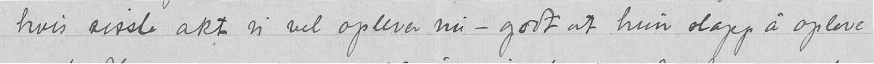hvis sisste akt vi vel oplever nu – godt at hun slapp å opleve
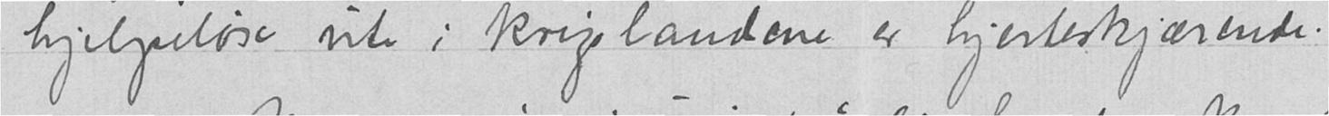hjelpeløse ute i krigslandene er hjerteskjærende.
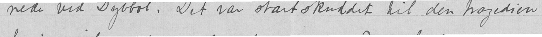nede ved Dybbøl. Det var startskuddet til den tragedien
